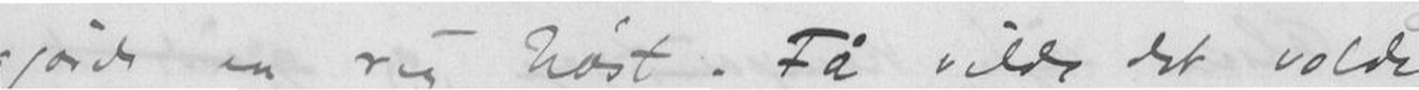gjorde en rig høst. Få vilde det volde
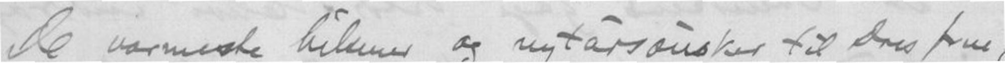De varmeste hilsener og nytårsønsker til Deres frue,
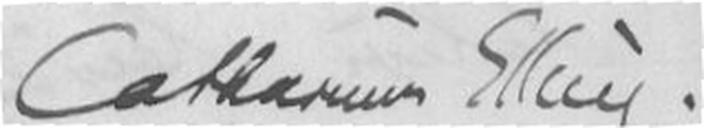Catharinus Elling
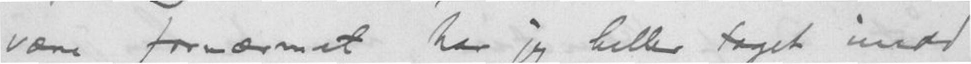være fornærmet har jeg heller taget imod
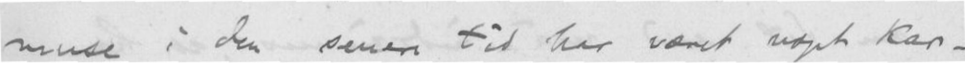muse i den senere tid har været noget kar-
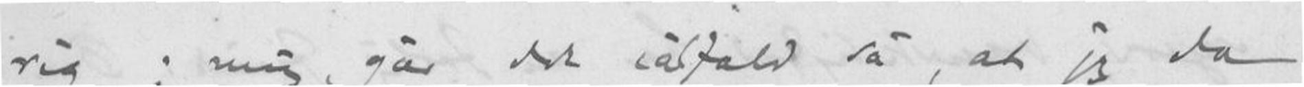rig; mig går det ialfald så, at jeg da
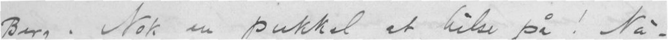Berg. Nok en pukkel at hilse på! Nå -
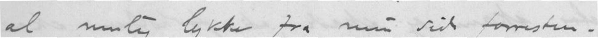al mulig lykke fra min side forresten.
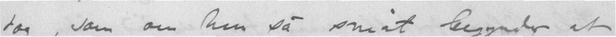dog, som om han så småt begynder at
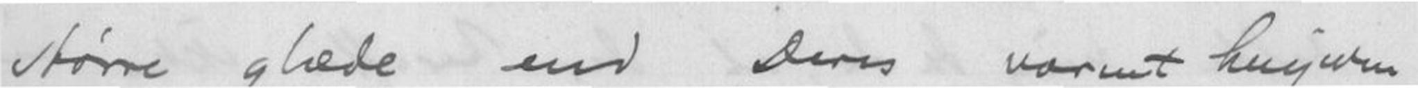støre glæde end Deres varmt hengivne
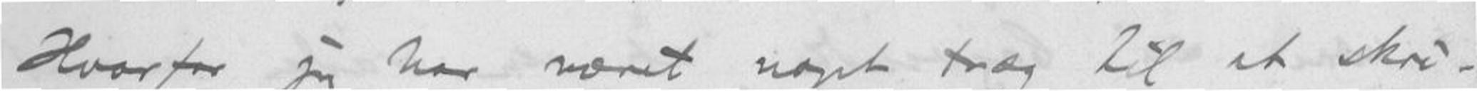Hvorfor jeg har været noget træg til at skri-
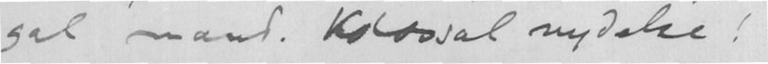gal mand. Kolosal nydelse!
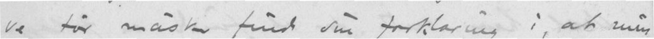ve tør måske finde sin forklaring i, at min
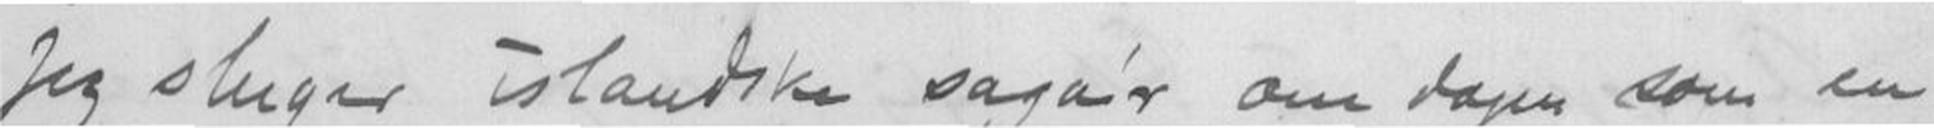Jeg sluget islandske saga'r om dagen som en
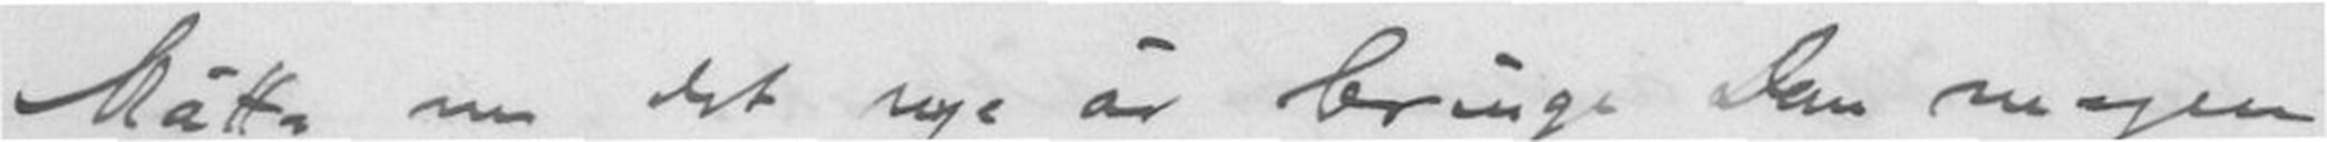Måtte nu det nye år bringe Dem megen
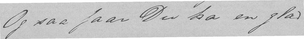Og saa faar Du ha en glad
